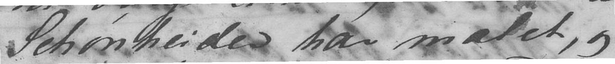Schønheider har malet, og
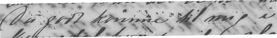Du godt komme til mig i
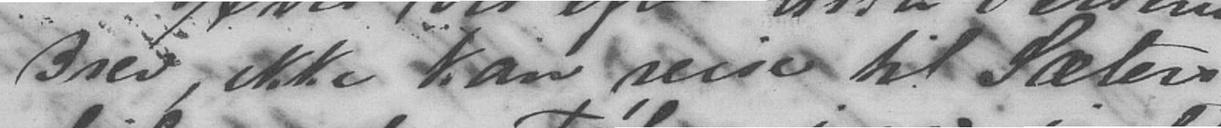Brev, ikke kan reise til Sæters-
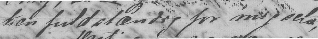ken fuldstændig for mig selv,
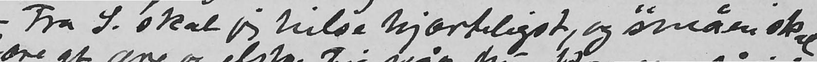-Fra S. skal jeg hilse hjærteligst, og småen skal
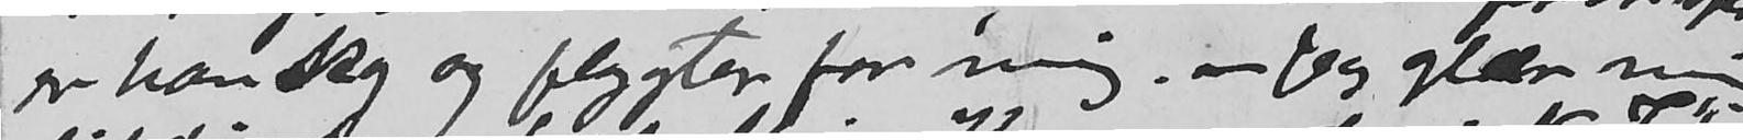er han sky og flygter for mig. -  Jeg  glæder mig
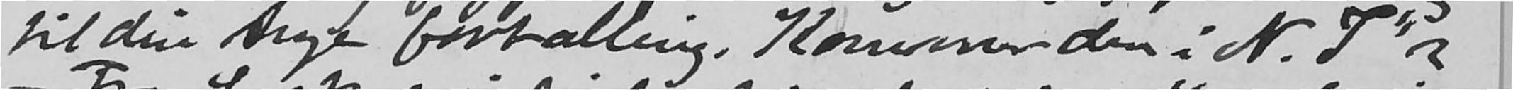til din nye fortælling. Kommer den: "N. T."?
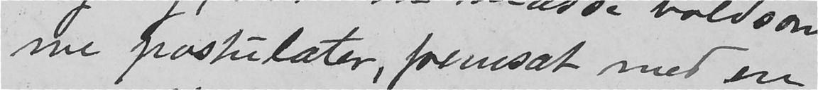me postulater, fremsat med en
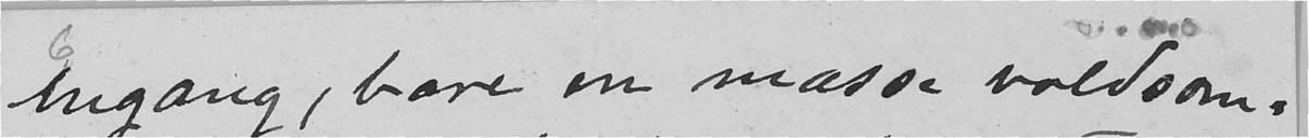engang, bare en masse voldsom-
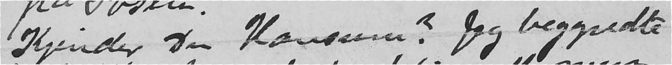Kjender Du Hansum? Jeg begyndte
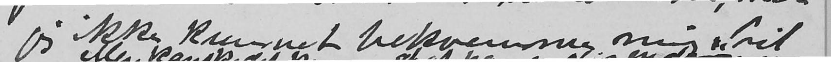jeg ikke kunnet bekvemme min til
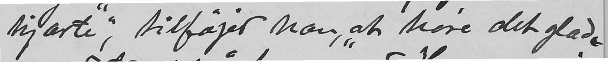hjærte," tilføjed han, "at høre det glade
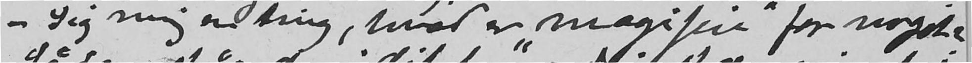- Sig mig en ting, hvad er "magisin" for noget?
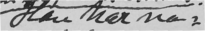Han har na-
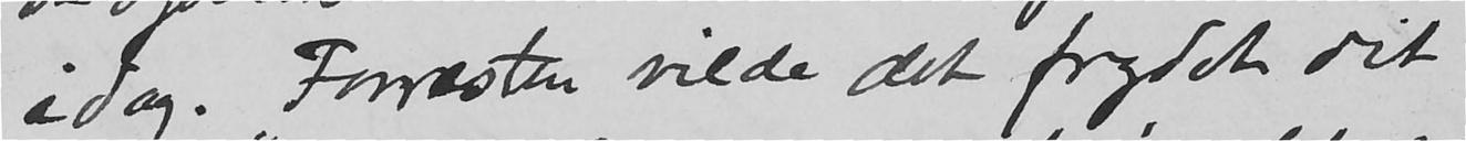idag. "Forresten vilde det frydet dit
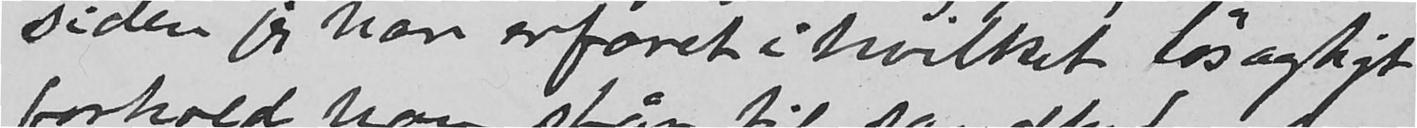siden jeg har erfaret i hvilket løsagtigt
